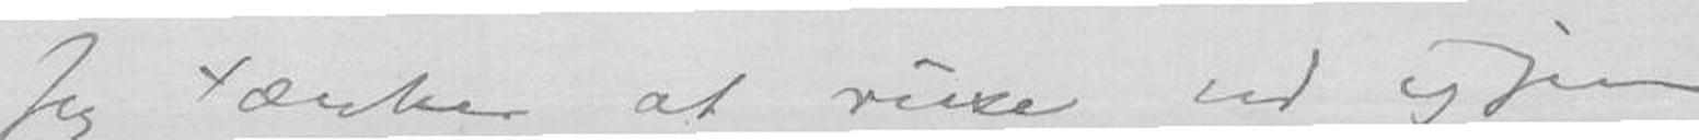Jeg tænker at reise ud igjen
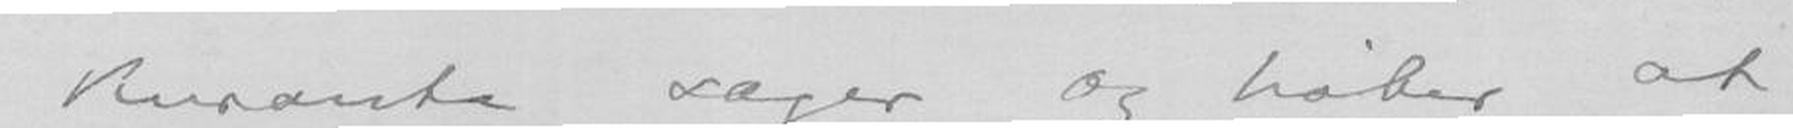kurante sager og håber at
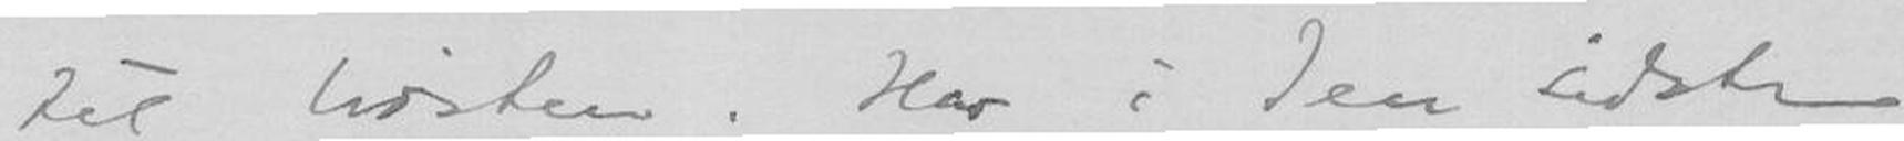til høsten. Har i den sidste
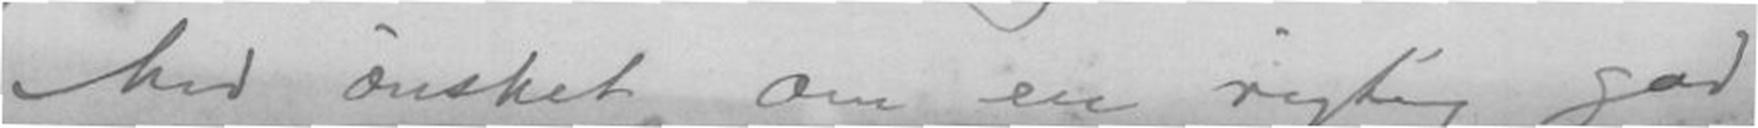Med ønsket om en rigtig god
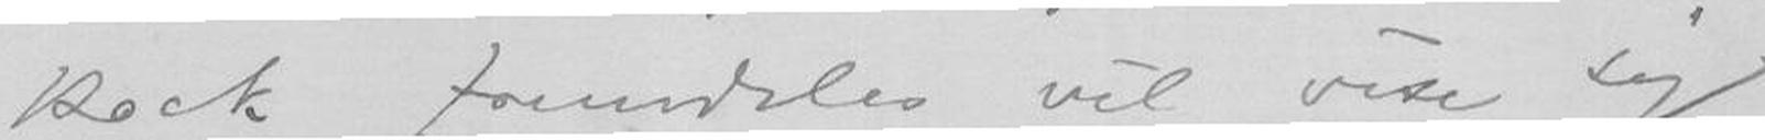Bock fremdeles vil vise sig
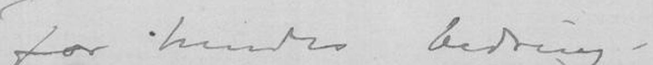for hendes bedring.
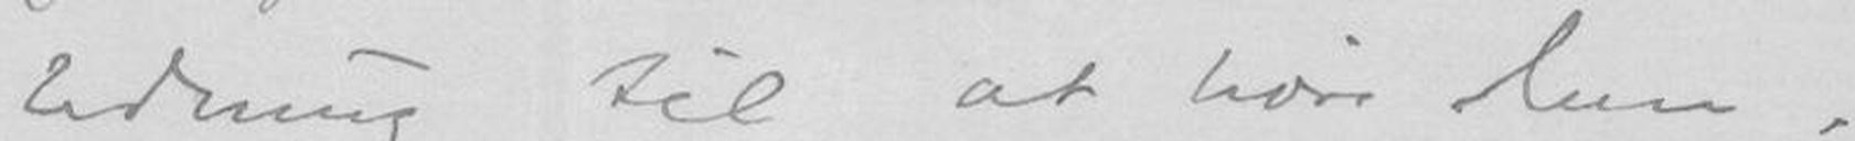ledning til at høre dem.
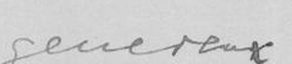genereux.
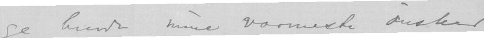ge hende mine varmeste ønsker
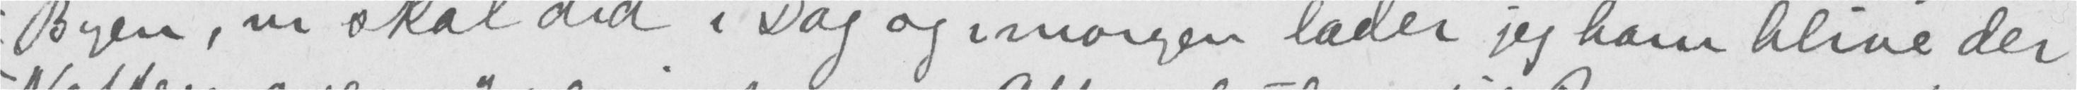Byen, vi skal did i Dag og imorgen lader jeg ham blive der
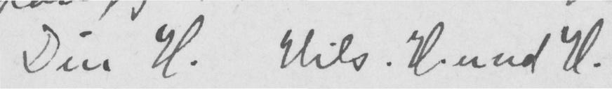Din H. Hils. H. und H.
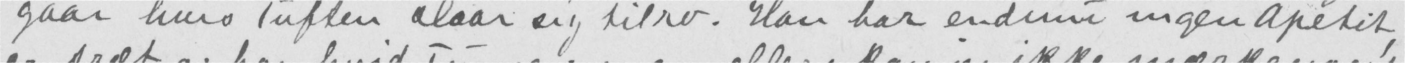gaar hvis Tuften slaar sig tilro. Han har endnu ingen apetit,
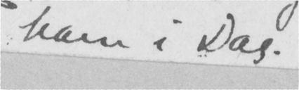ham i Dag.
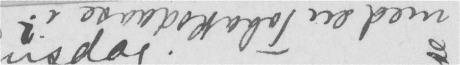med en tobakdaase i?
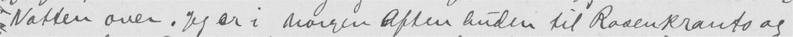natten over. Jeg er i morgen aften buden til Rosenkranto og
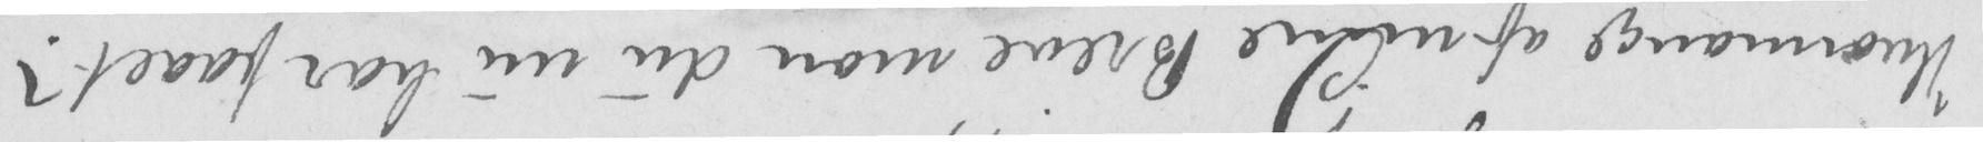Hvormange af mine breve mon du nu har faaet?
